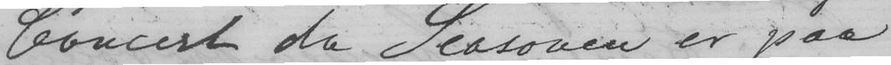Concert da Seasonen er paa
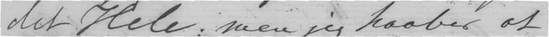det Hele; men jeg haaber at
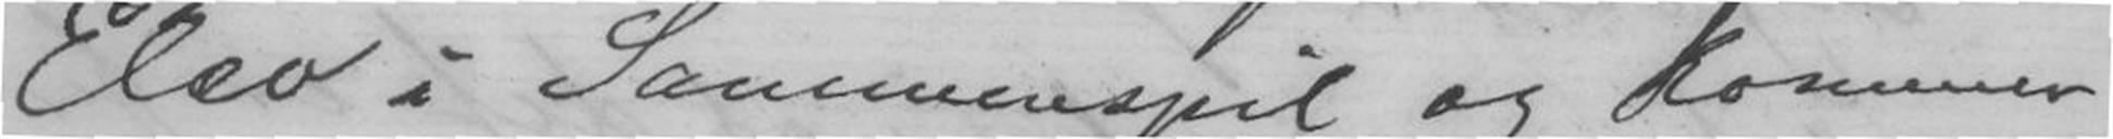Elev i Sammenspil og kommer
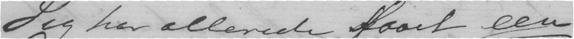Jeg har allerede faaet een
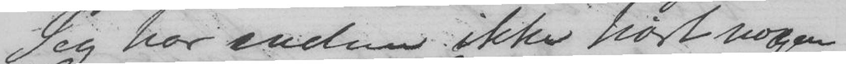Jeg har endnu ikke hørt nogen
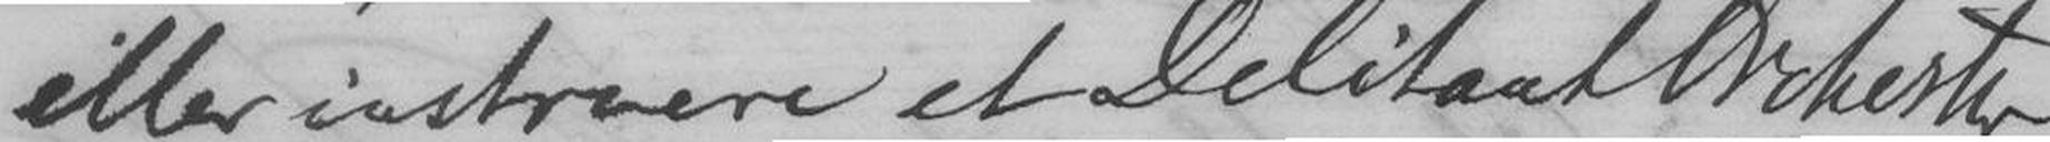eller instruere et Dilitant Orkester
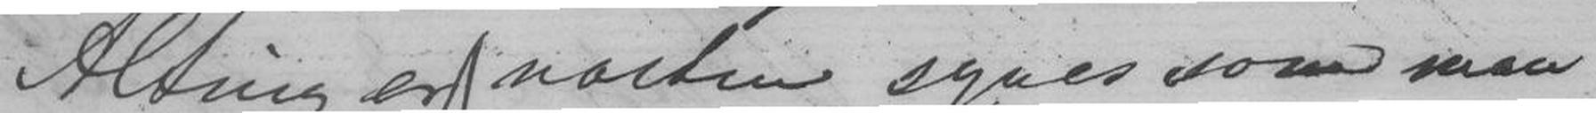Alting er næsten synes som man
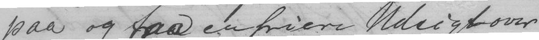paa og faa enfriere Udsigt over
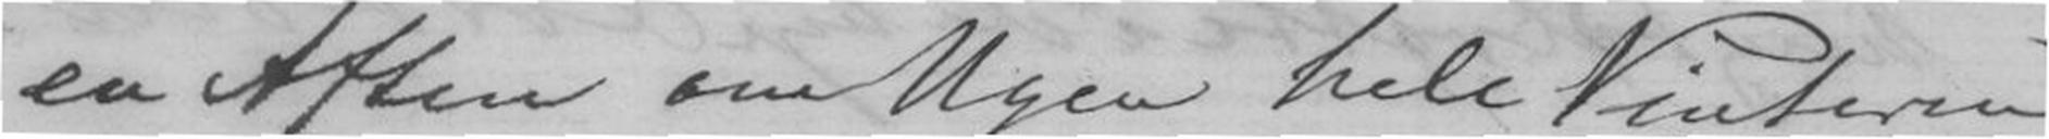en Aften om Ugen hele Vinteren
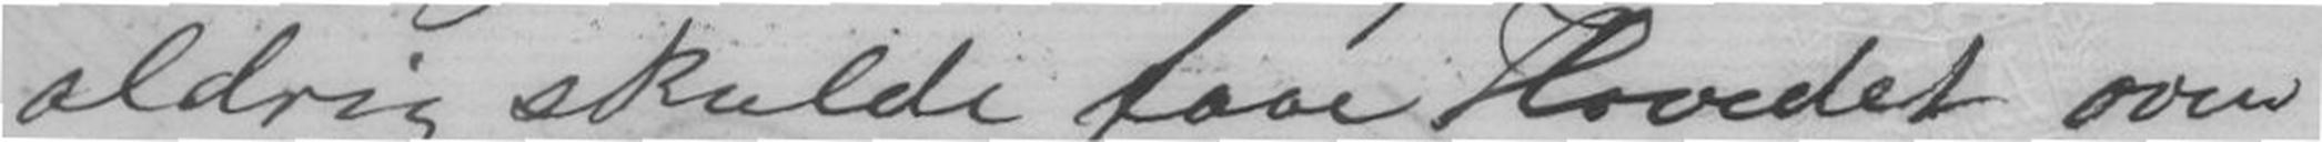aldrig skulde faae Hovedet oven
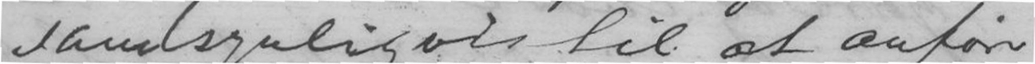sandsynligvis til at anføre
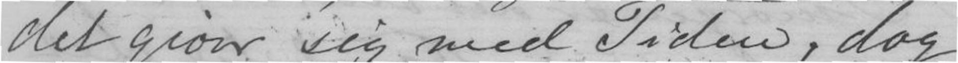det giver sig med Tiden, dog
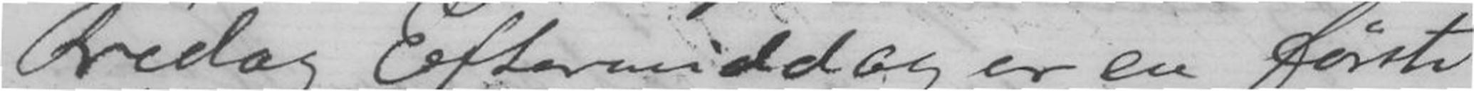Fredag Eftermiddag er en første
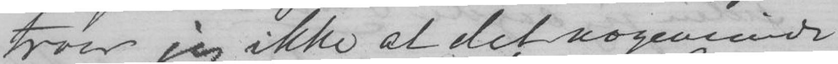troer jeg ikke at det nogensinde
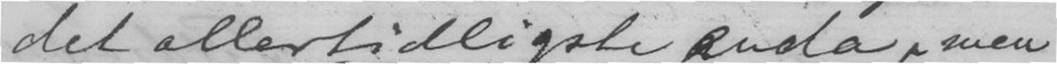det allertidligste enda, men
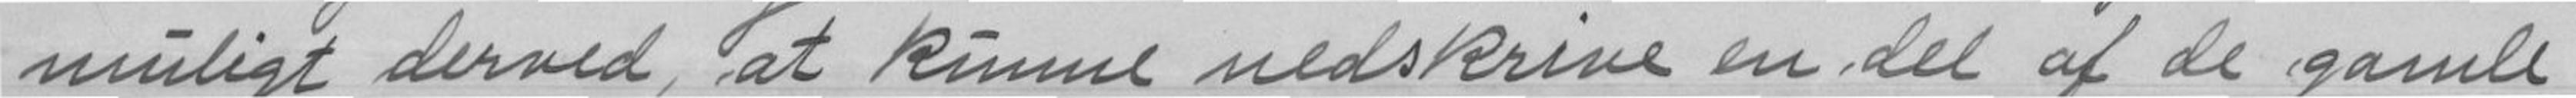muligt derved at kunne nedskrive en del af de gamle
